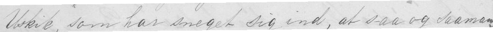Uskik, som har sneget sig ind, at saa og saa man-
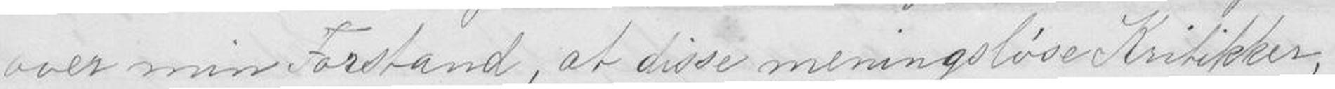over min Forstand, at disse meningsløse Kritikker,
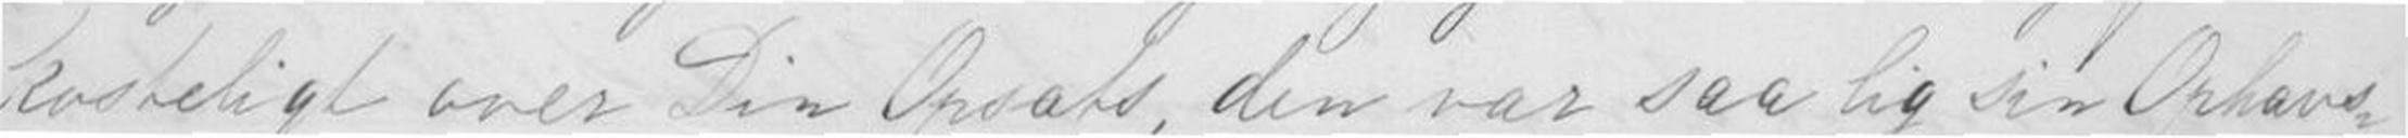kosteligt over Din Opsats, den var saa lig sin Ophavs-
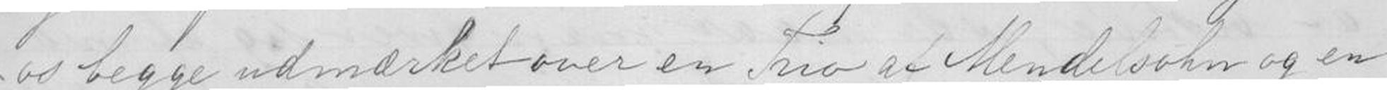os begge udmærket over en Tio af Mendelsohn og en
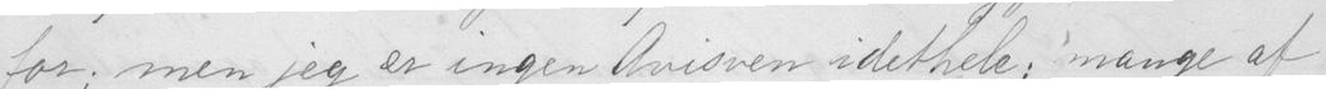for; men jeg er ingen Avisven idethele; mange af
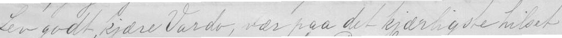Lev godt, kjære Vardo, vær paa det kjærligste hilset
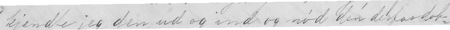kjendte jeg den ud og ind og nød den derfo dob-
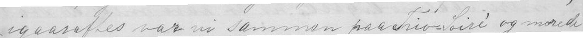igaaraftes var vi sammen paa Tio-Soiré og morede
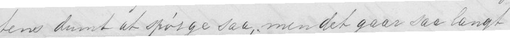tens dumt at spørge saa, men det gaar saa langt
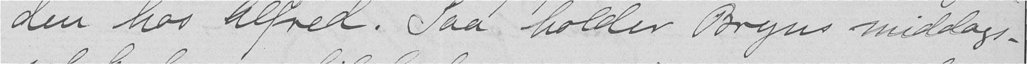den hos Alfred. Saa holder Bryns middags-
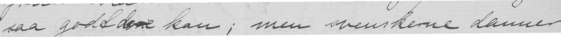saa godt dere kan; men svenskerne danner
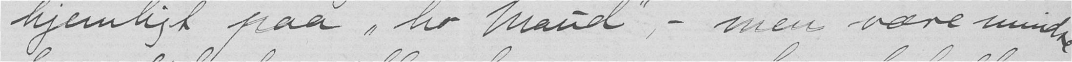hjemligt paa "ho Maud", - men være mindre
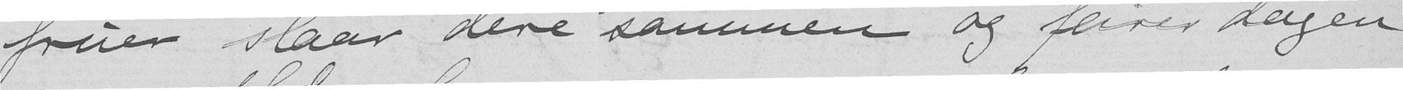fruer slaar dere sammen og feirer dagen
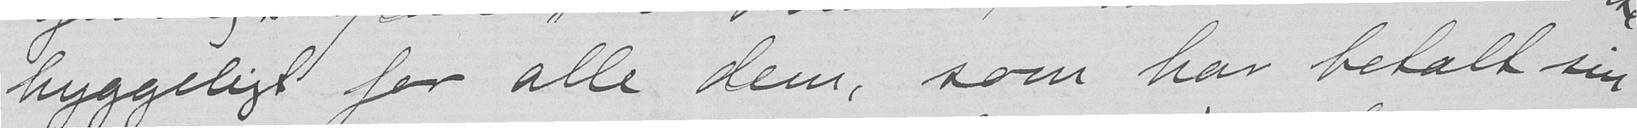hyggeligt for alle dem, som har betalt sin
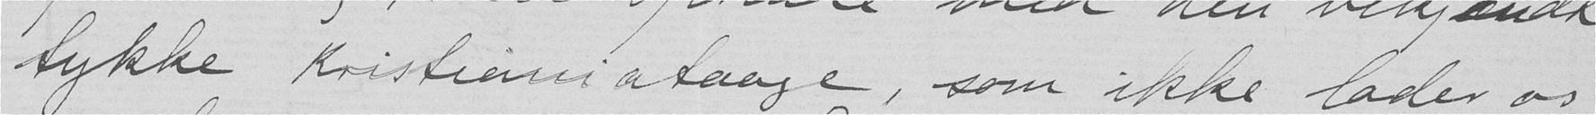tykke Kristianiataage, som ikke lader os
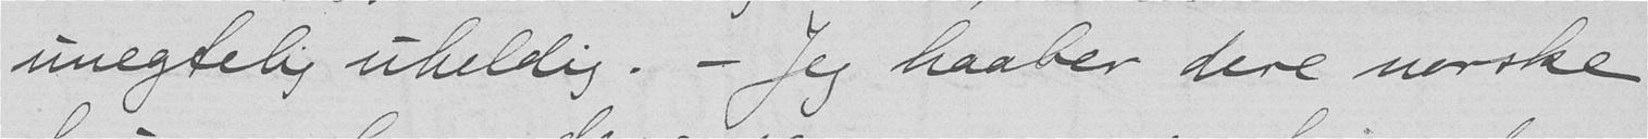unegtelig uheldig. - Jeg haaber dere norske
